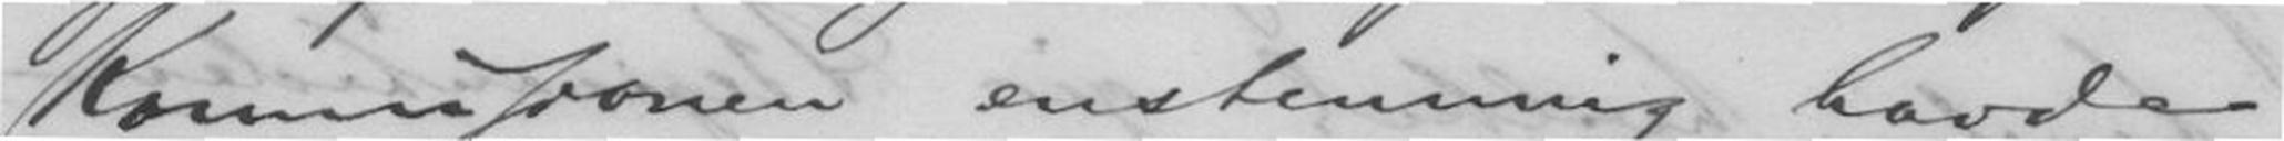Kommissionen enstemmig havde
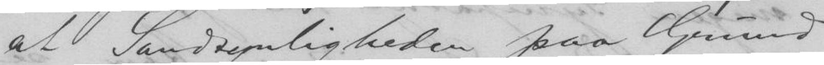at Sandsynligheden paa Grund
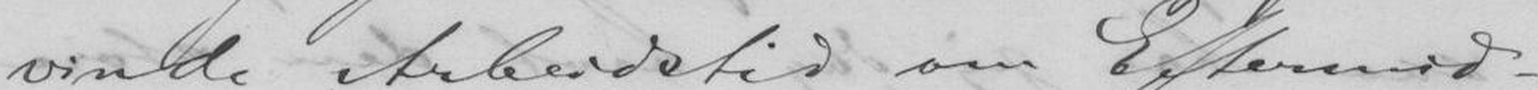vinde Arbeidstid om Eftermid-
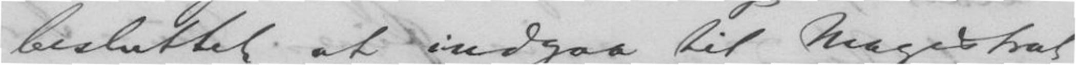besluttet at indgaa til Magistrat
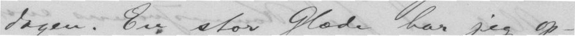dagen. En stor Glæde har jeg op-
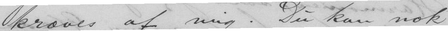kræves af mig. Du kan nok
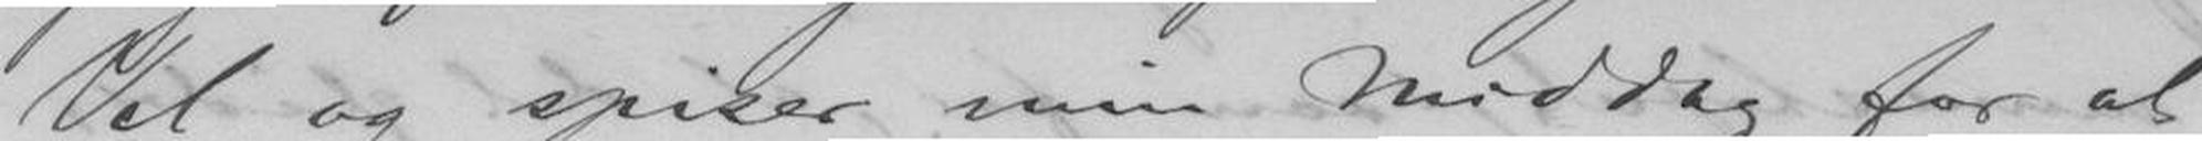Vel og spiser min Middag for at
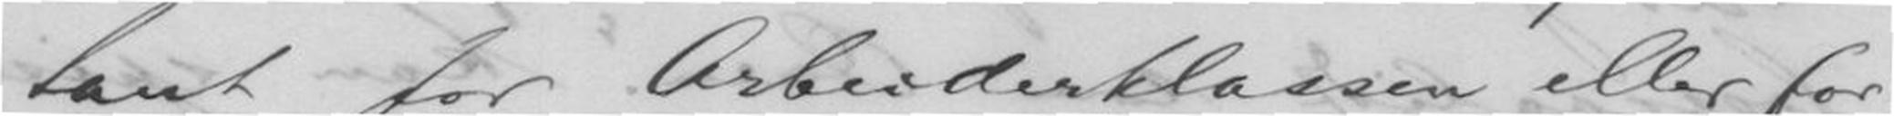tant for Arbeiderklassen eller for
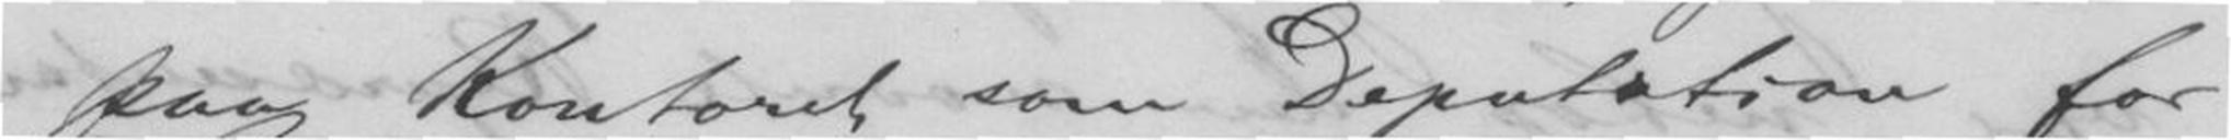paa Kontoret som Deputation for
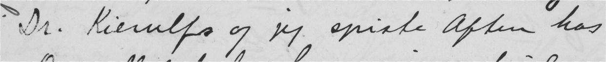Dr. Kierulfs og jeg spiste Aften hos
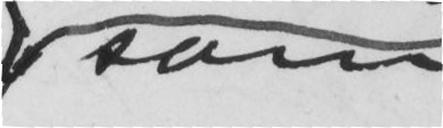som
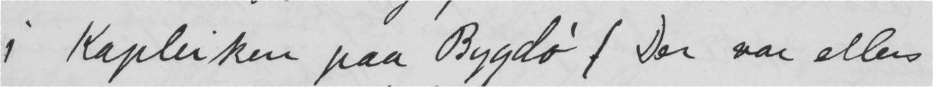i Kapleiken paa Bygdø (Der var ellers
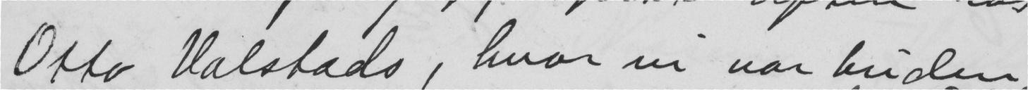Otto Valstads, hvor vi var buden
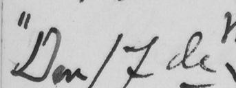"Den 17de
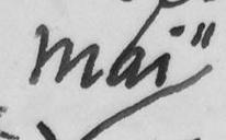mai"
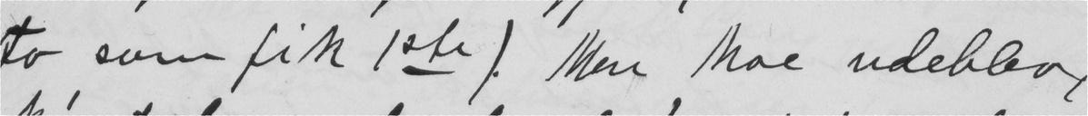to som fik 1ste). Men Moe udeblev,
